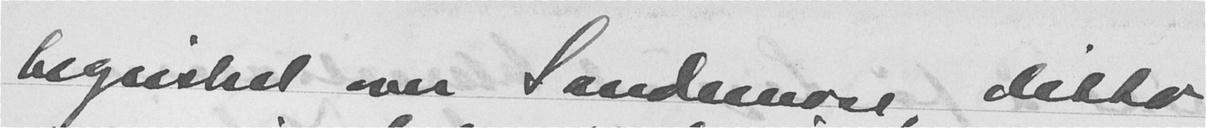begeistret over Sandemose, ditto
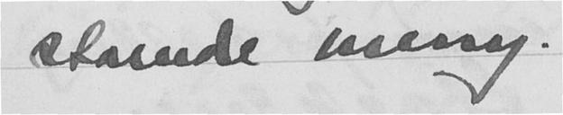starnde usersy.
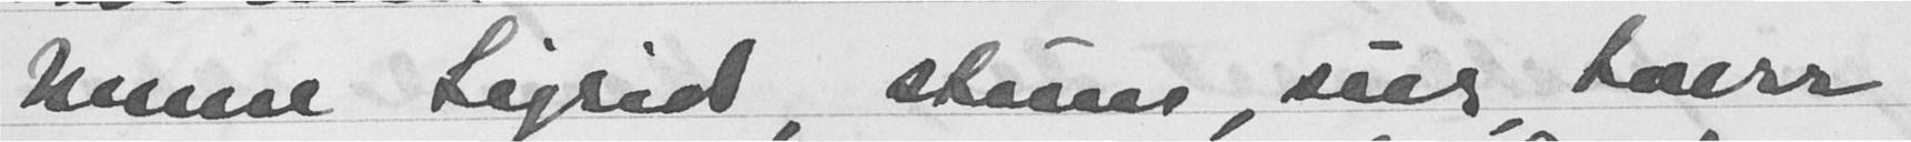kunne Sigrid, stum, sur, trass
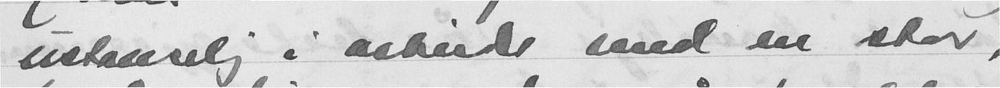ustanselig i arbeide med en stor,
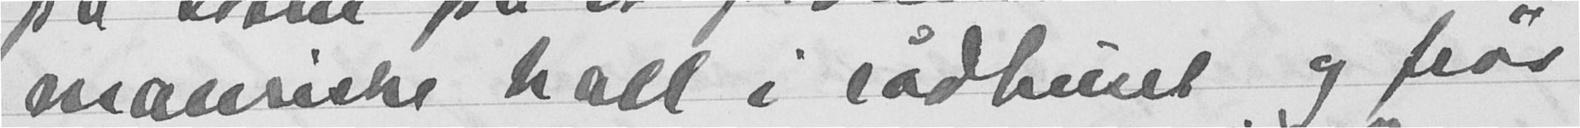mansiske hall i rådhuset og slår
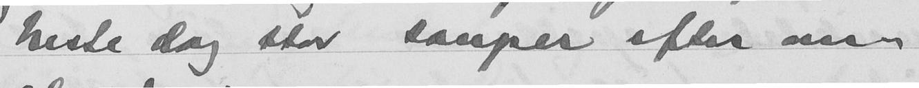Neste dag stor souper efter om
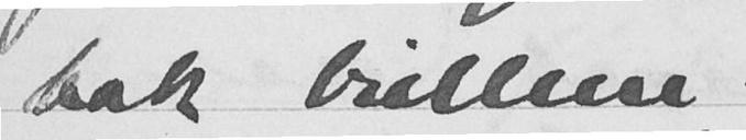bak brillene.
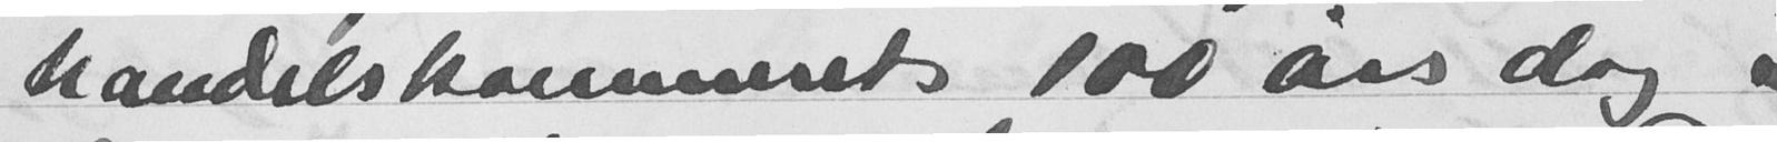handelskomusets 100 års dag.
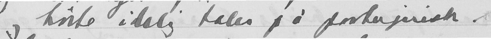og hørte idelig taler på portugusisk.
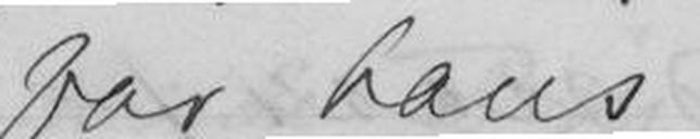for hans
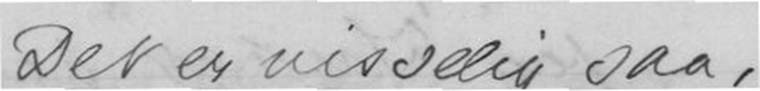Det er visselig saa,
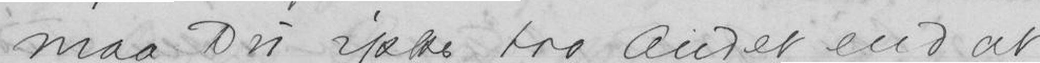maa Du ikke tro Andet end at
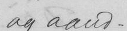og aand-
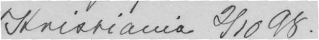Kristiania 2/10 98
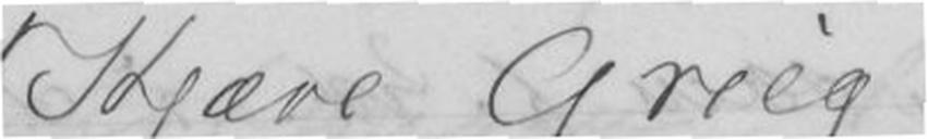Kjære Grieg
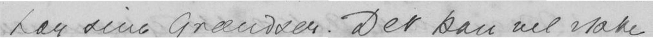har sine Grændser. Det kan vel ikke
